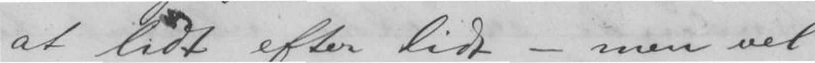at lidt efter lidt - men vel
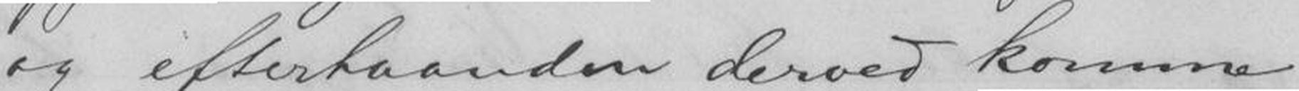og efterhaanden derved komme
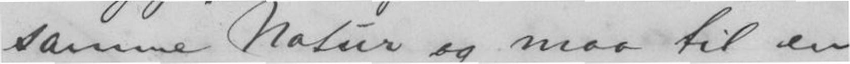samme Natur og maa til en
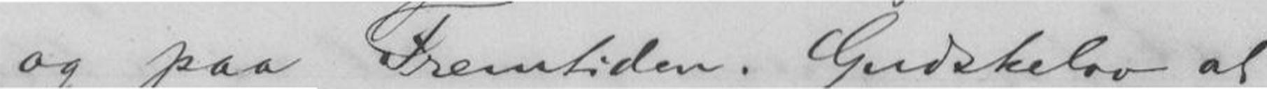og paa Fremtiden. Gudskelov at
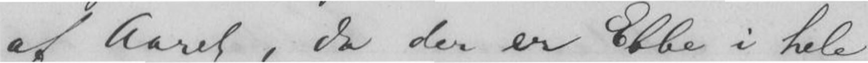af Aaret, da der er Ebbe i hele
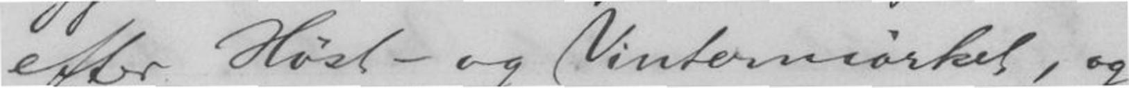efter Høst- og Vintermørket, og
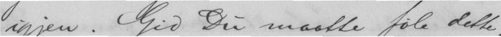igjen. Gid Du maatte føle dette,
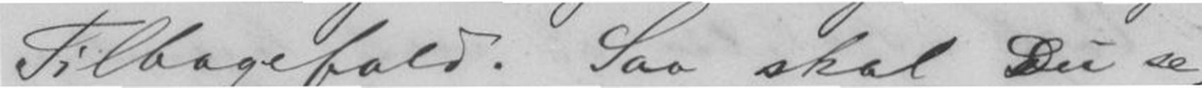Tilbagefald. Saa skal Du se,
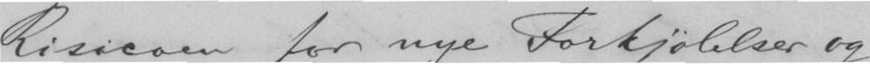Risicoen for nye Forkjølelser og
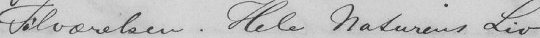Tilværelsen. Hele Naturens Liv
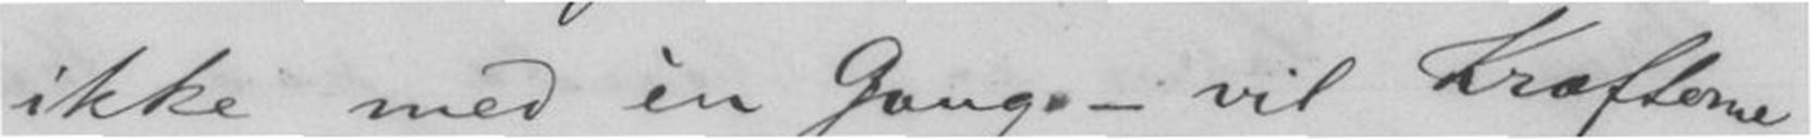kke med èn Gang - vil Kræfterne
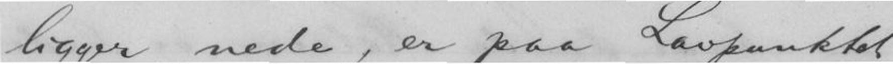ligger nede, er paa Lavpunktet
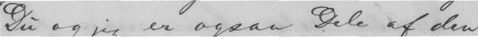Du og jeg er ogsaa Dele af den
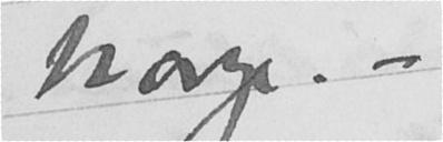Norge. -
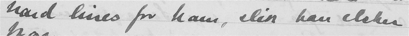hard liner for ham, slik han elsker
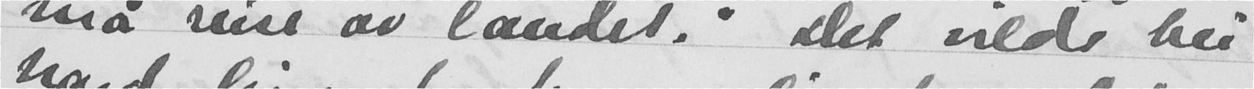må reise av landet." Det vilde bli
